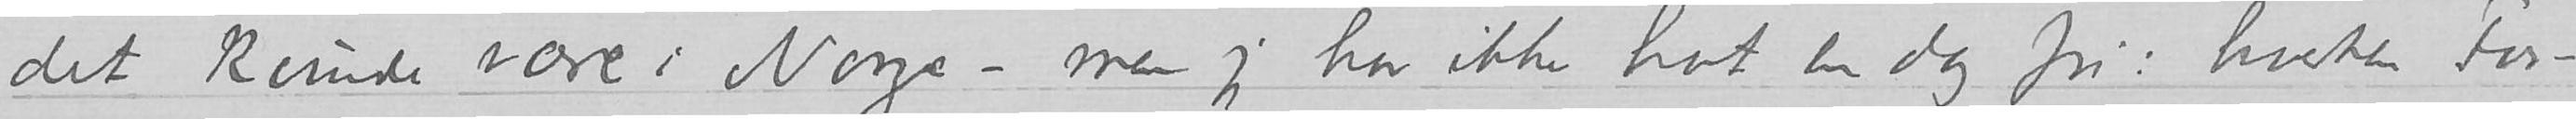det kunde være i Norge – men jeg har ikke hat en dag fri: hverken For-
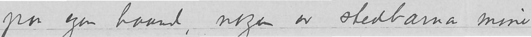paa egen haand, nogen av stedbarna mine.
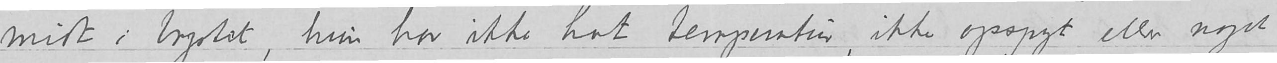midt i brystet, hun har ikke hat temperatur, ikke opspyt eller noget
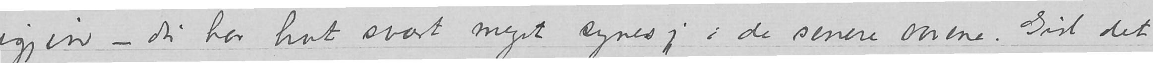igjen – du har hat svært meget synes jeg i de senere aarene. Gid det
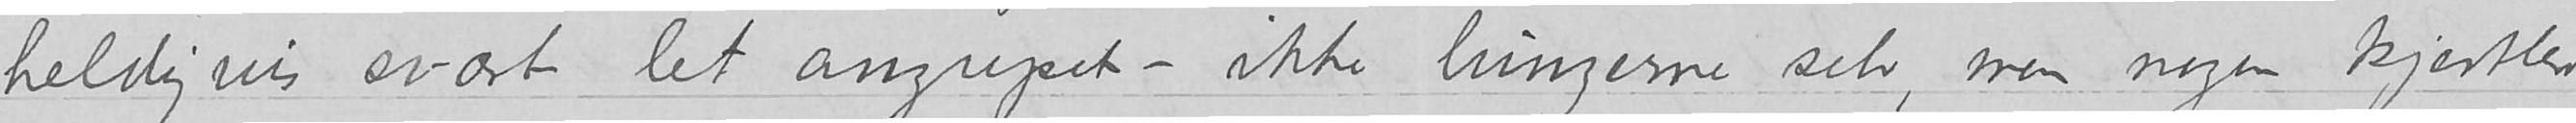heldigvis svært let angrepet – ikke lungerne selv, men nogen kjertler
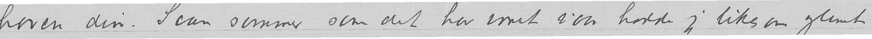haven din. Saan sommer som det har været iaar hadde jeg liksom glemt
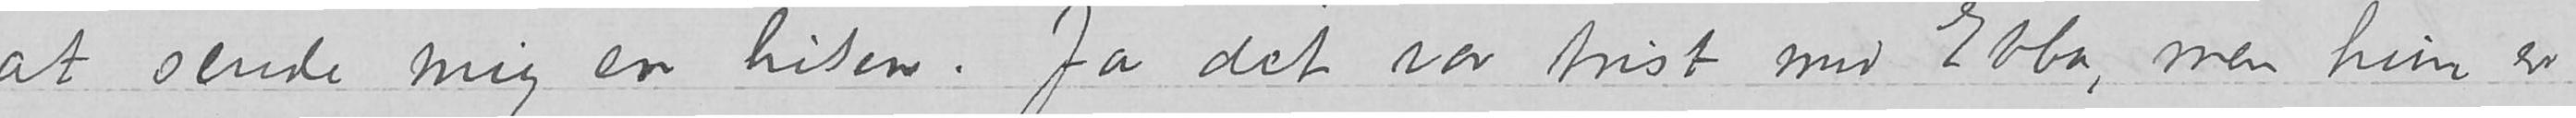at sende mig en hilsen. Ja det var trist med Ebba, men hun er
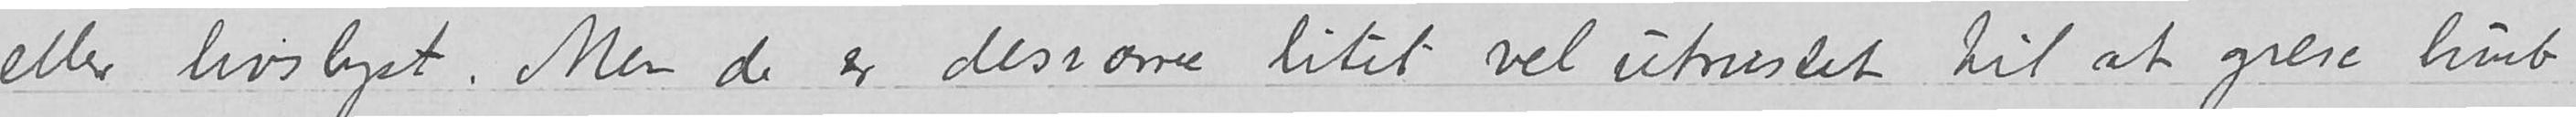eller livslyst. Men de er desværre litet vel utrustet til at greie livet
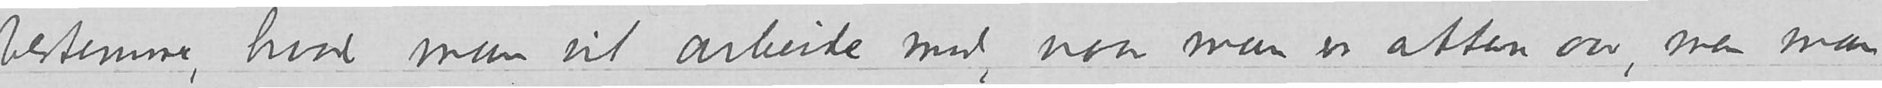bestemme, hvad man vil arbeide med, naar man
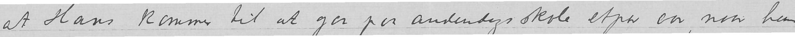at Hans kommer til at gaa paa andendagsskole etpar aar, naar han nu
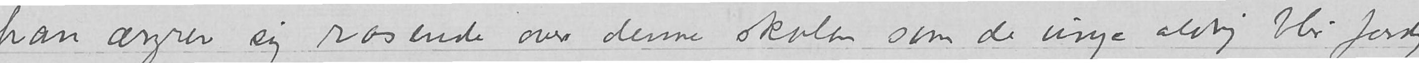han ærgrer sig rasende over denne skolen som de unge aldrig blir færdig
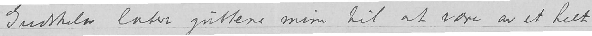Gudskelov later gutterne mine til at være av et helt
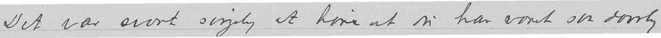Det var svært sørgelig at høre at du har været saa daarlig
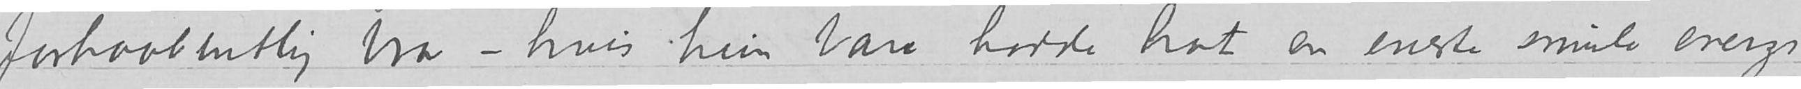forhaabentlig bra – hvis hun bare hadde hat en eneste smule energi
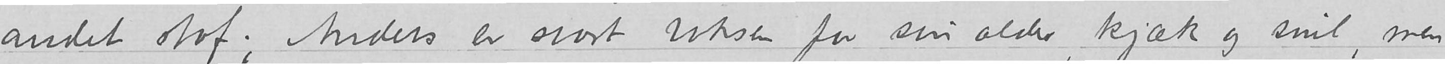andet stof; Anders er svært voksen for sin alder, kjæk og snil, men
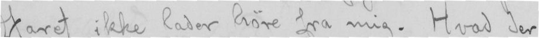Aaret ikke lader høre fra mig. Hvad der
